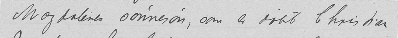Magdalenes sønnesøn, som er døbt Christian
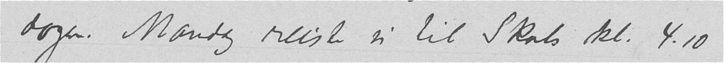dagen. Mandag reiste vi til Skals kl. 4.10
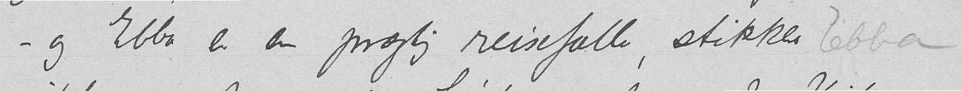– og Ebba er en prægtig reisefælle, stikker
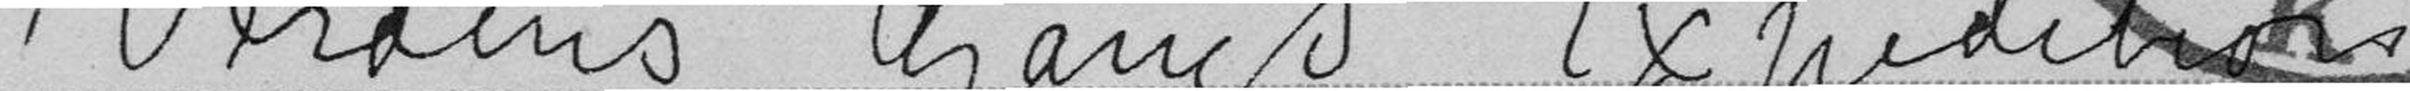Verdens Gangs Expedition
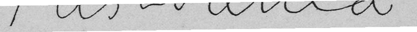Kristiania
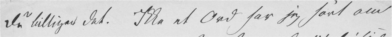Du billiger det. Ikke et Ord har jeg hørt om
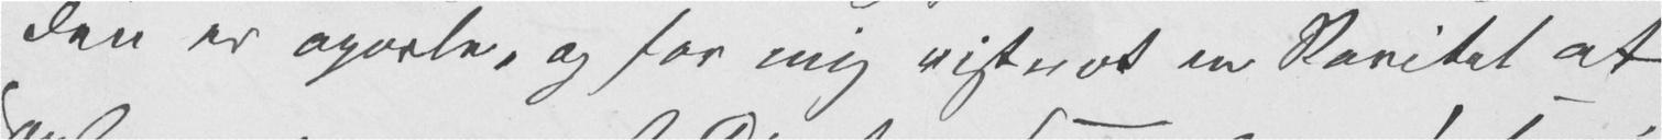den er aparte, og for mig vistnok en Raritet at
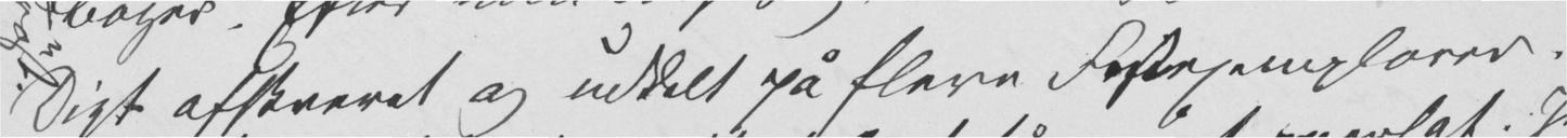Digt afskrevet og uddelt på flere Frstexemplarer.
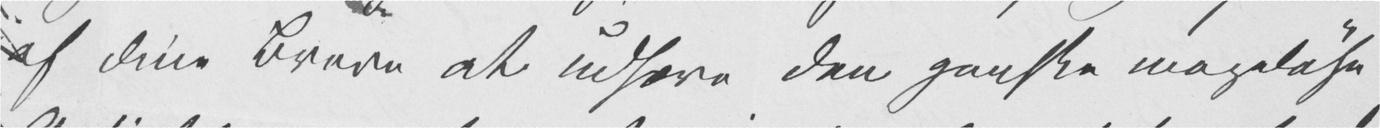af Dine Breve at udhæve den ganske mageløse
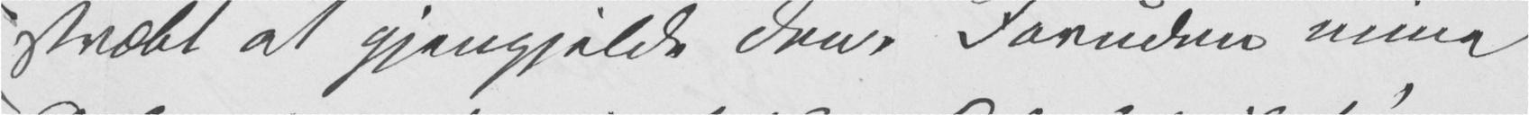stræbt at gjengjelde den, Foruden mine
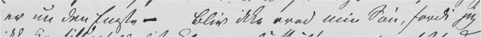er nu den Eneste – Bliv ikke vred min Søn, fordi jeg
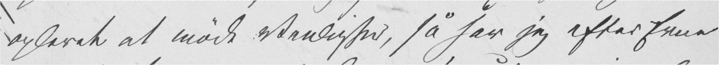oplevet at møde Venlighed, så har jeg efter Evne
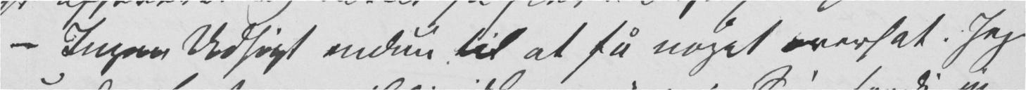– Ingen Udsigt endnu til at få noget overfat. Jeg
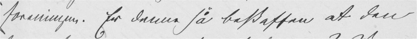foreningen. Er denne så beskaffen at den
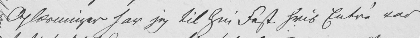Oplæsninger har jeg til hin Fest hvis Entré var
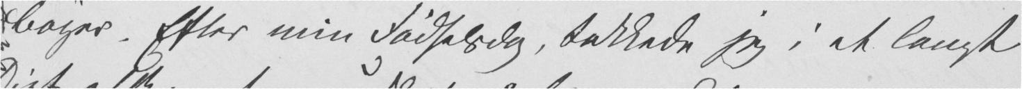Bøger. Efter min Fødselsdag, takkede jeg i et langt
